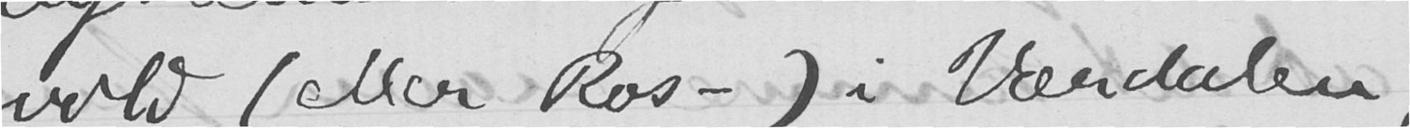vold (eller Ros-) i Værdalen,
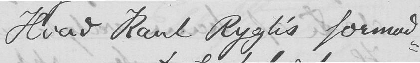Hvad Karl Ryghs formod-
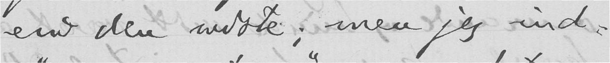end den sidste; men jeg ind-
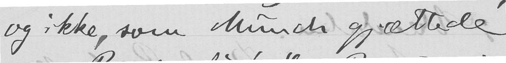og ikke, som Munch gjættede
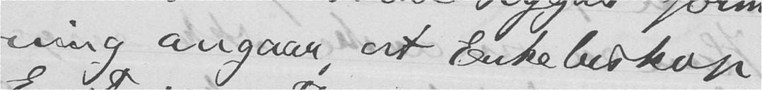ning angaar, at Erkebiskop
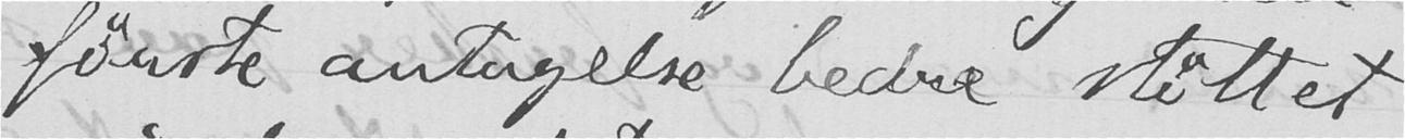første antagelse bedre støttet
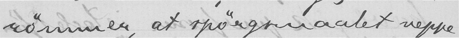rømmer at spørgsmaalet neppe
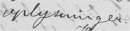oplysninger.
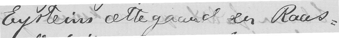Eysteins ættegaard er Raas-
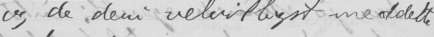og de deri velvilligst meddelte
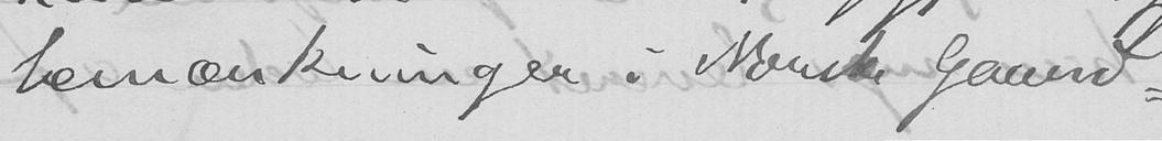bemærkninger i Norske Gaard-
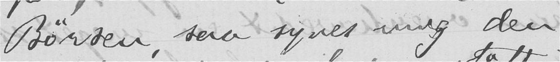Børsen, saa synes mig den
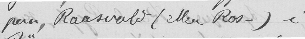paa, Raasvold (eller Ros-) i
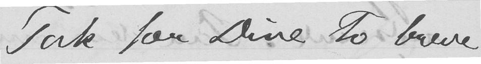Tak for Dine to breve
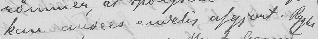kan ansees endelig afgjort. Ryghs
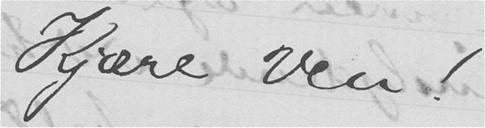Kjære ven!
